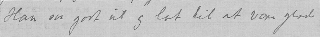Han saa godt ut og lot til at være glad
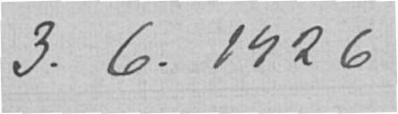3.6.1926
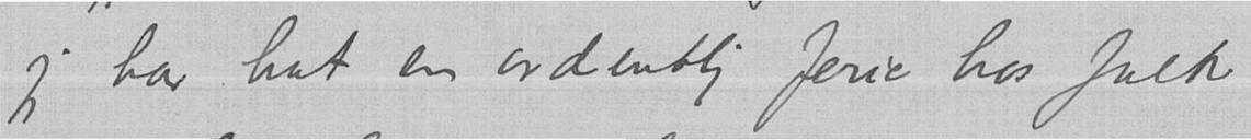jeg har hat en ordentlig ferie hos folk
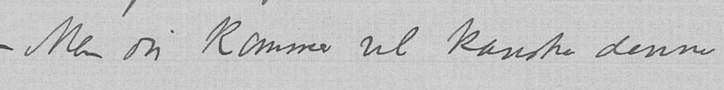- Men du kommer vel kanske denne
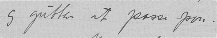og gutten at passe paa.
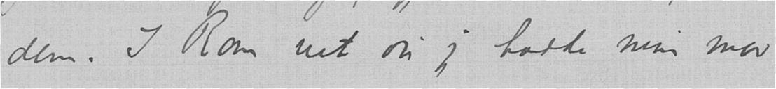dem. I Rom vet du jeg hadde min mor
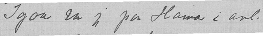Igaar var jeg paa Hamar i anl.
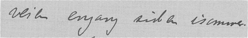veien engang siden isommer.
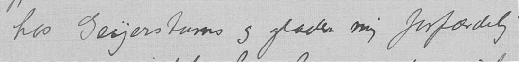hos Geijerstams og glæder mig forfærdelig
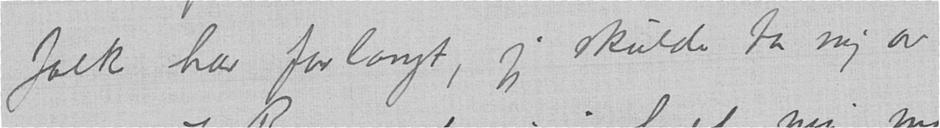folk har forlangt, jeg skulde ta mig av
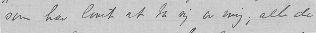som har lovet at ta sig av mig; alle de
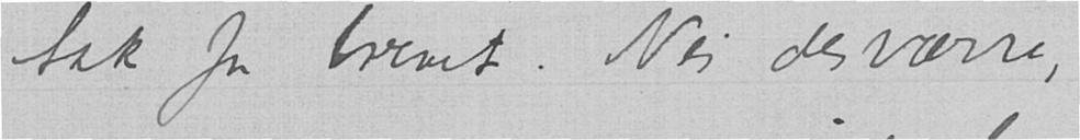tak for brevet. Nei desværre,
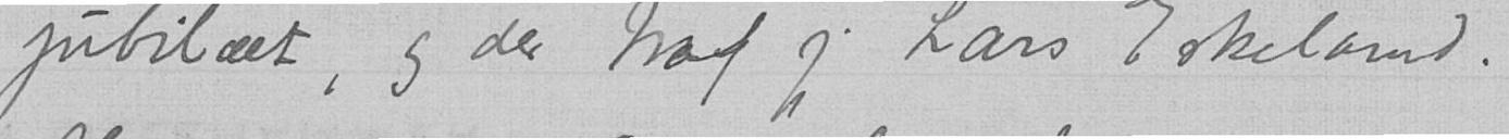jubilæet, og der traf jeg Lars Eskeland.
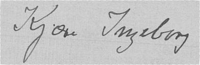Kjære Ingeborg,
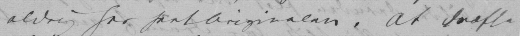aldrig har seet Originalen, At træffe
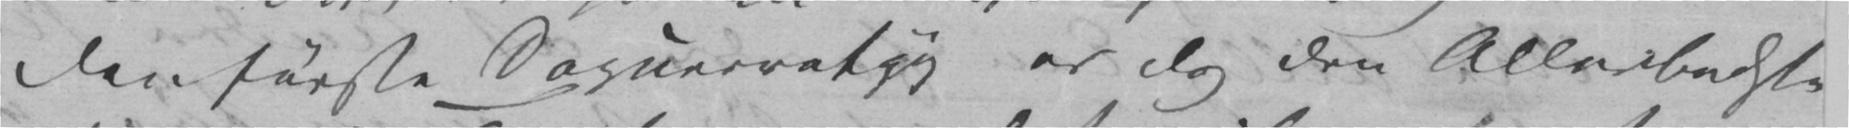Den første Daguerrotyp er dog den Allerbedste
